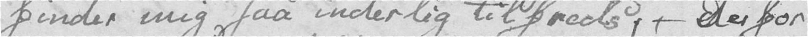finder mig saa inderlig tilfreds, – derfor
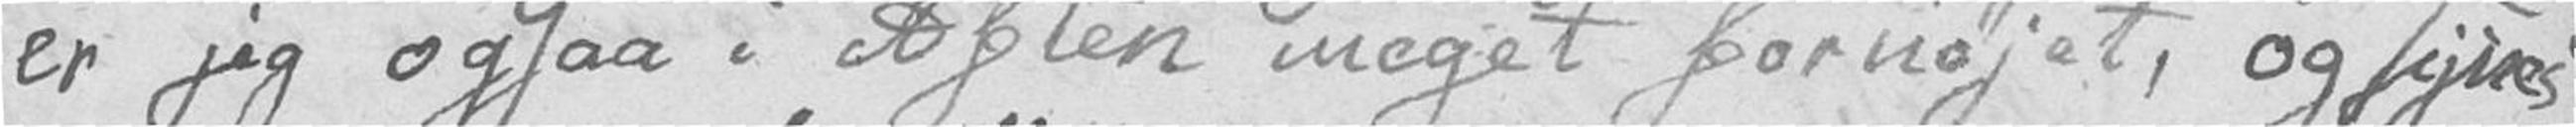er jeg ogsaa i Aften meget fornøjet, og synes
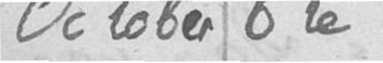October 6te
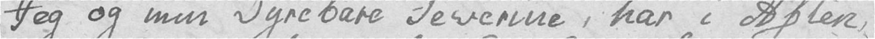Jeg og min Dyrebare Severine, har i Aften,
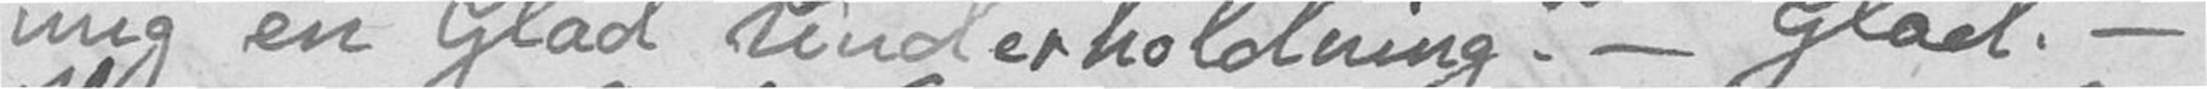mig en Glad Underholdning. – Glad. –
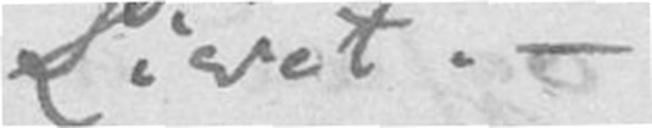Livet. –
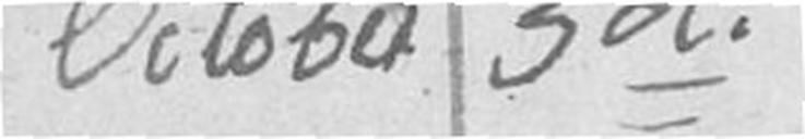October 3di
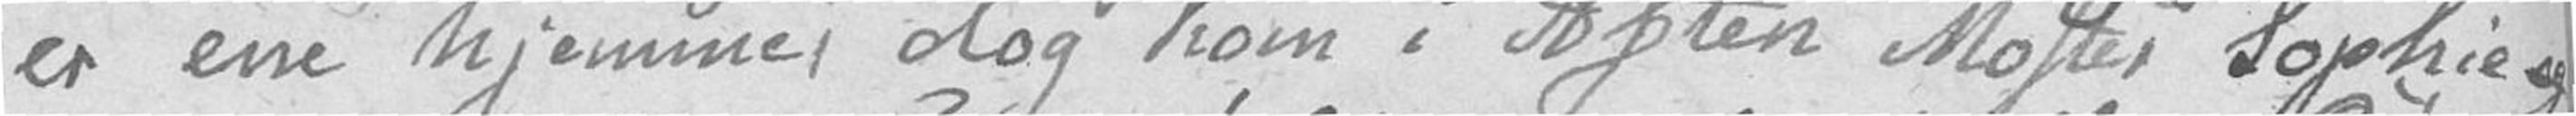er ene hjemme, dog kom i Aften Moster Sophie og
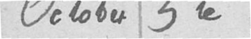October 5te
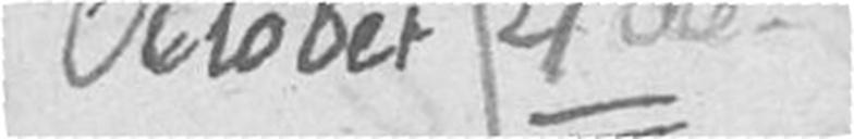October 4de
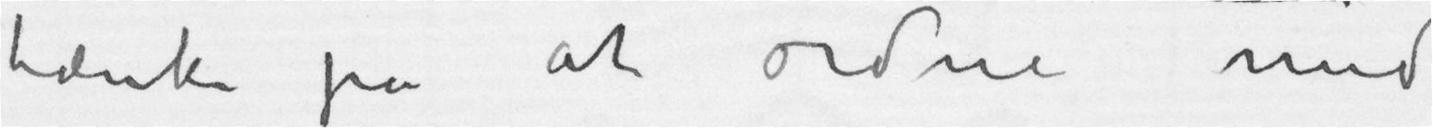tænke på at ordne med
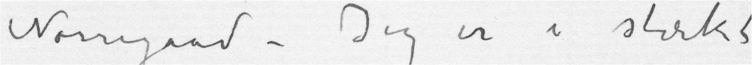Nørregaard – Jeg er i stærkt
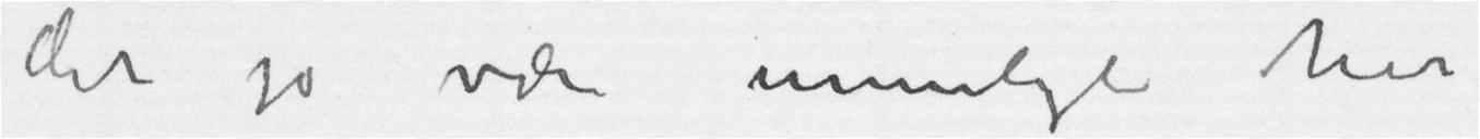det jo være umuligt her
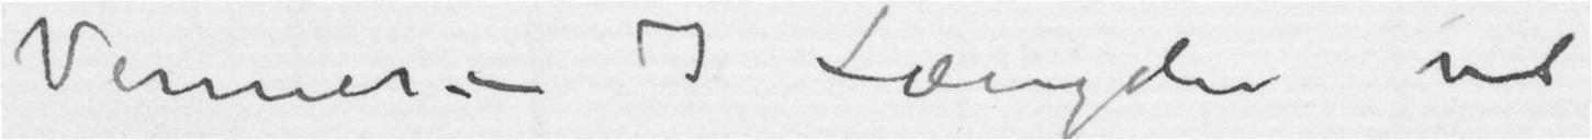Venner – I længden vil
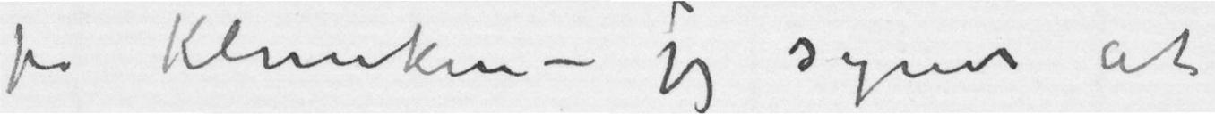på Klinikken – Jeg synes at
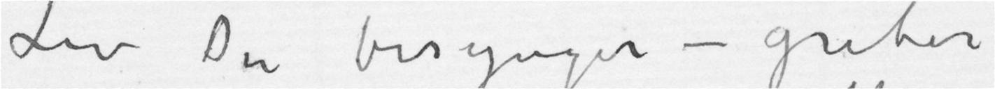Liv Du besynger – griber
